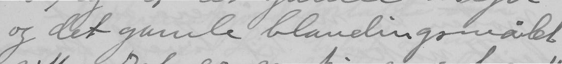og det gamle blandingsmålet
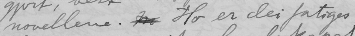novellene. Ho er dei fatiges
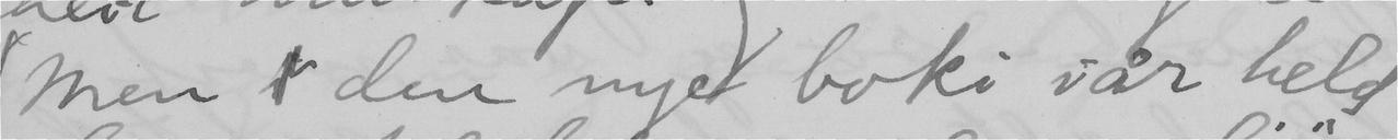Men den nye boki iår held
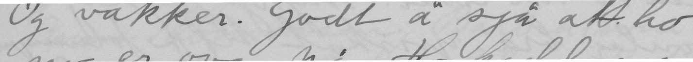og vakker. Godt å sjå at ho
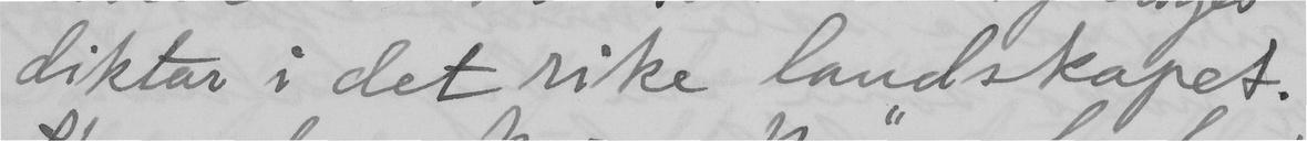diktar i det rike landskapet.
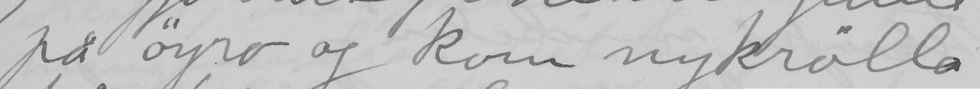på öyro og kom nykrölla
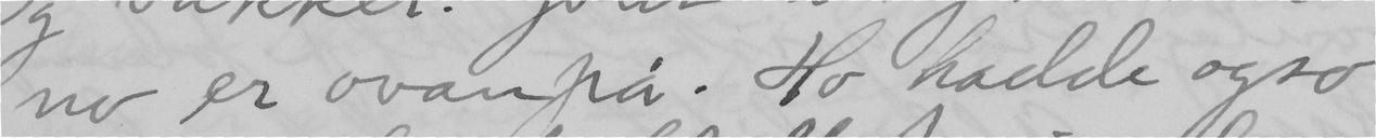no er ovanpå. Ho hadde ogso
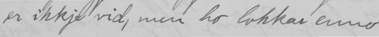er ikkje vid, men ho lokkar enno
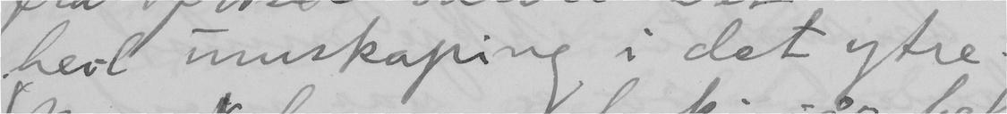heil umskaping i det ytre.
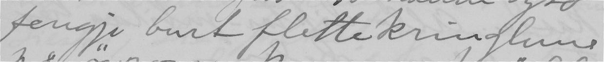fengje burt flettekringlane
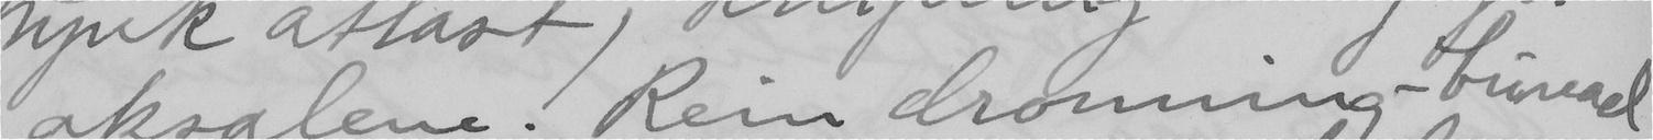aksalene. Rein dronning-bunad
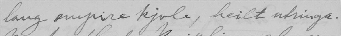lang empire kjole, heilt utringa.
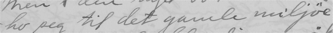ho seg til det gamle miljöe
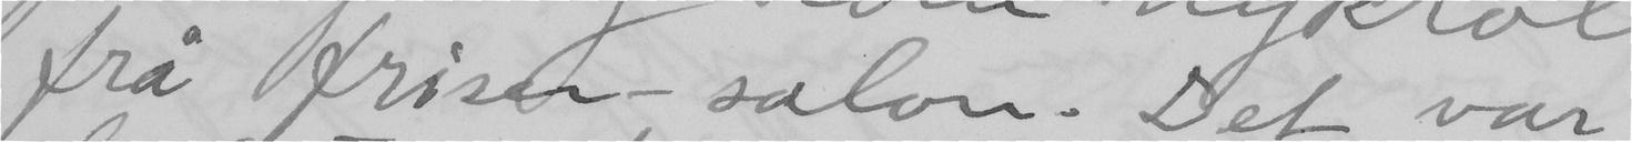frå friser-salon. Det var
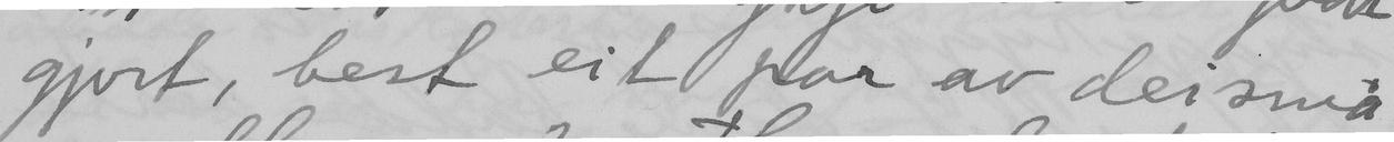gjort, best eit par av dei små
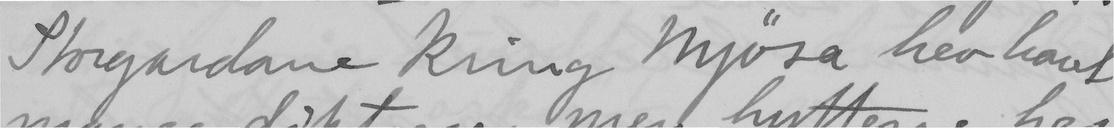Storgardane kring Mjösa hev havt
